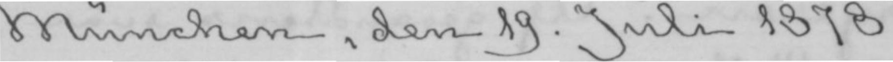München, den 19. Juli 1878.
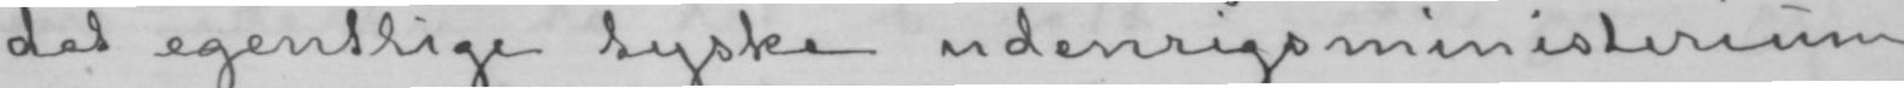det egentlige tyske udenrigsministerium
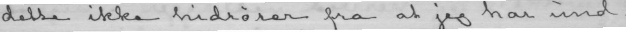dette ikke hidrører fra at jeg har und-
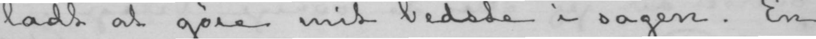ladt at gøre mit bedste i sagen. En
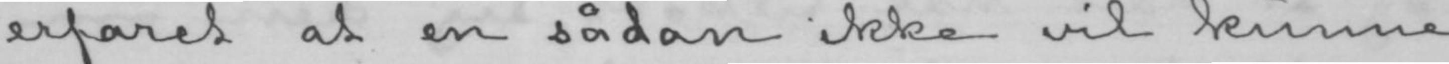erfaret at en sådan ikke vil kunne
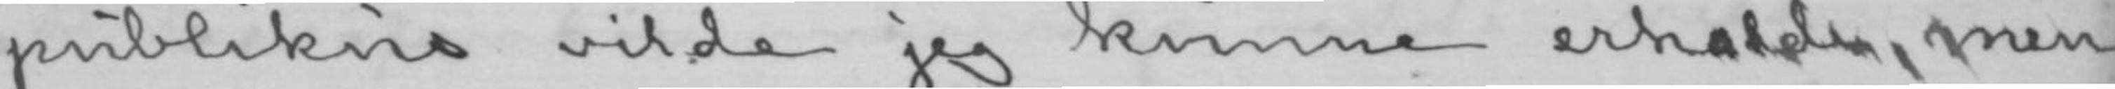publikus vilde jeg kunne erholde, men
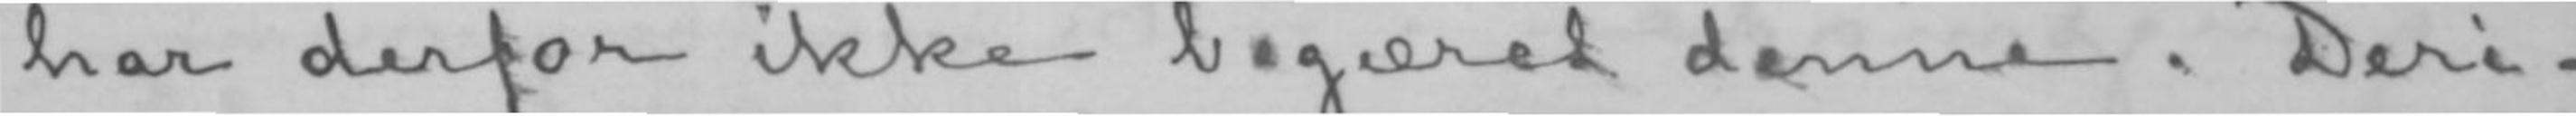har derfor ikke begæret denne. Deri-
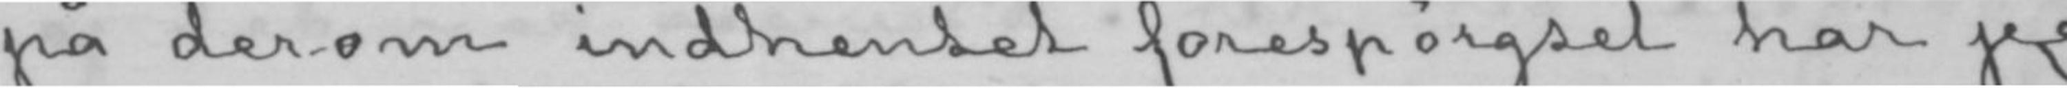på derom indhentet forespørgsel har jeg
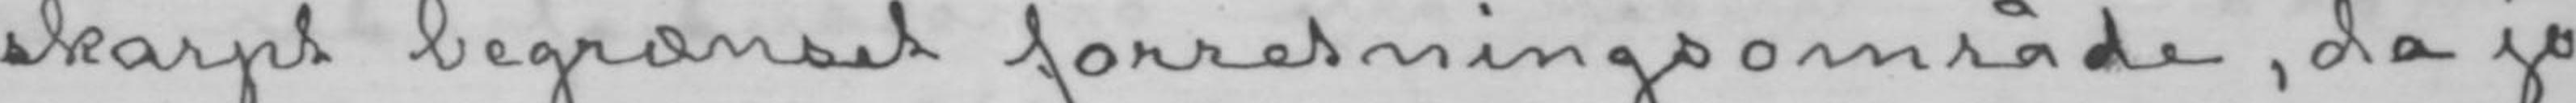skarpt begrænset forretningsområde, da jo
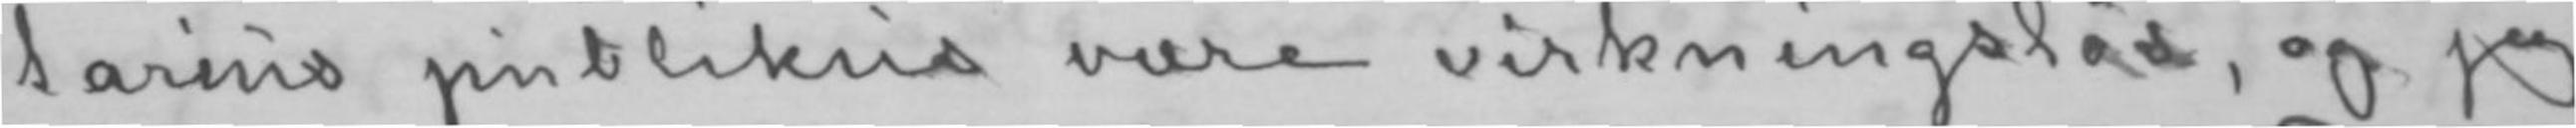tarius publikus være virkningsløs, og jeg
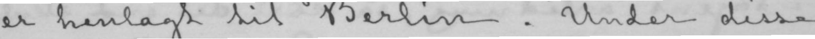er henlagt til Berlin. Under disse
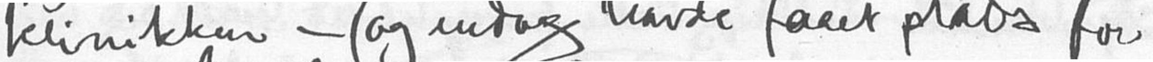klinikken - (og endog havde faaet plads for
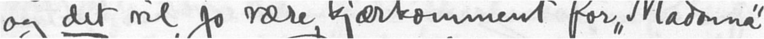og det vil jo være kjærkomment for "Madonna"
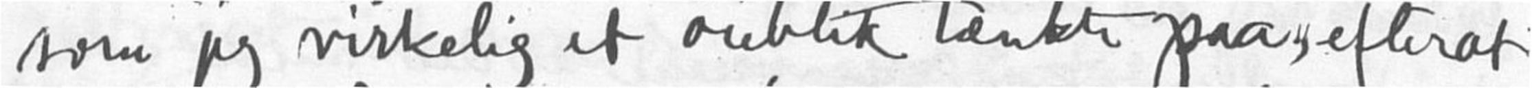som jeg virkelig et øieblik tænkte paa, efterat
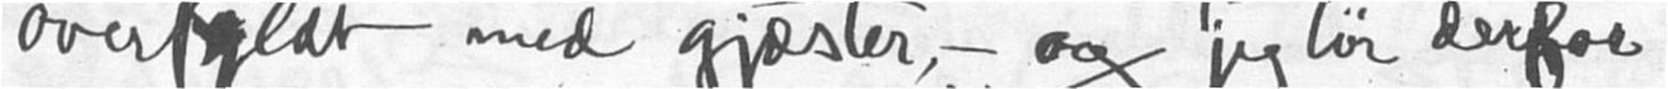overfyldt med gjæster, - og jeg tør derfor
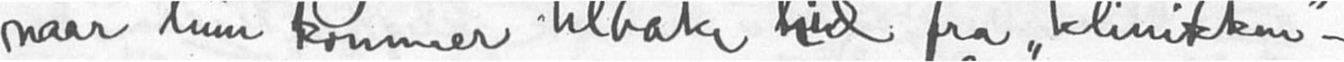naar hun kommer tilbake hid fira "klinikken" -
